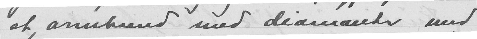at armbaand med diamanter med
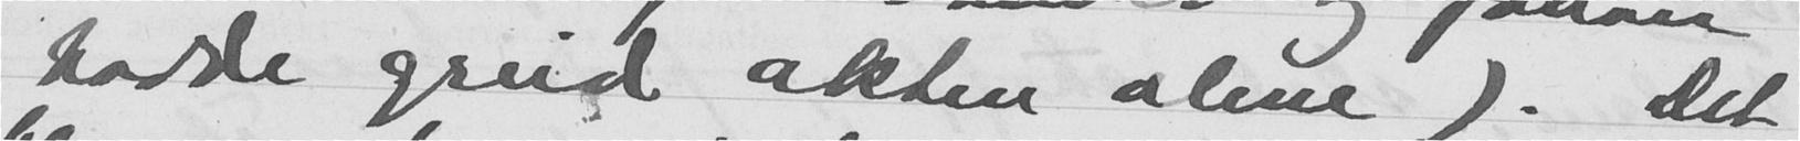hadde greid akten alene). Det
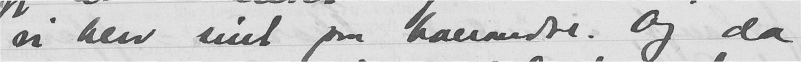vi blev vist paa hanvedte. Og da
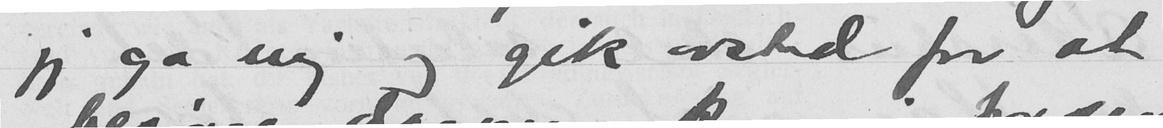jeg ga mig og gik avsted for at
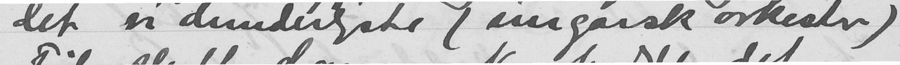det vidunderligste (ungarsk orkester)
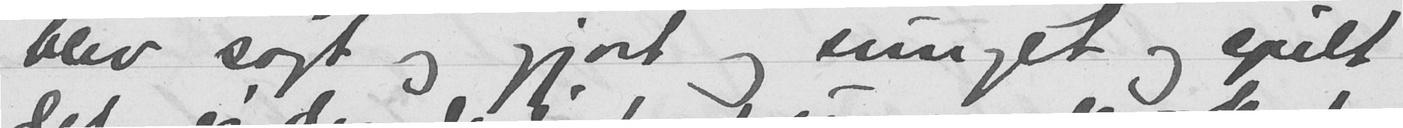blev sagt og gjort og sunget og spilt
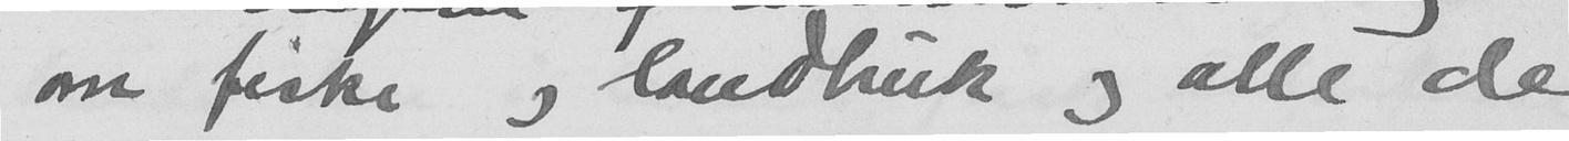om fiske og landbruk og alle de
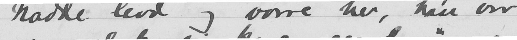hadde levd og vorre her, han var
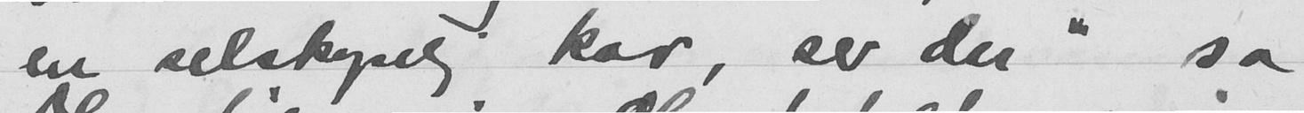en selskapelig kar, ser du" sa
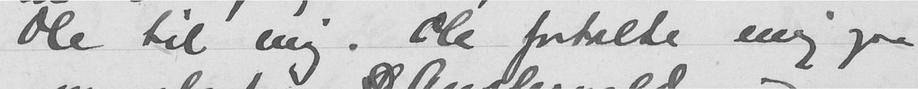Ole til mig. Ole fortalte mig saa
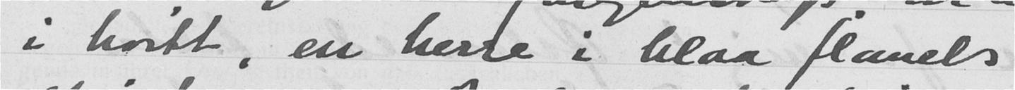i høitt, en herre i blaa flanels
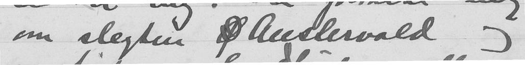om slegten Ø Austervold og
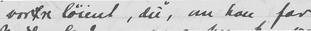vortre løient, du, om han far
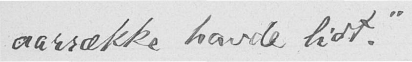aarrække havde lidt."
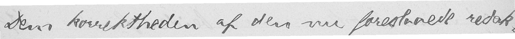Dem korrektheden af den nu foreslaaede redak-
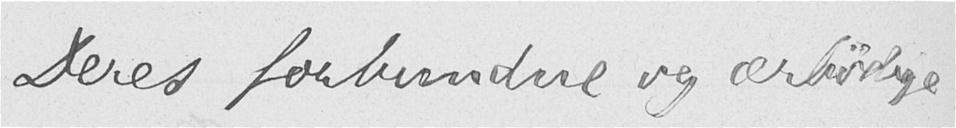Deres forbundne og ærbødige
